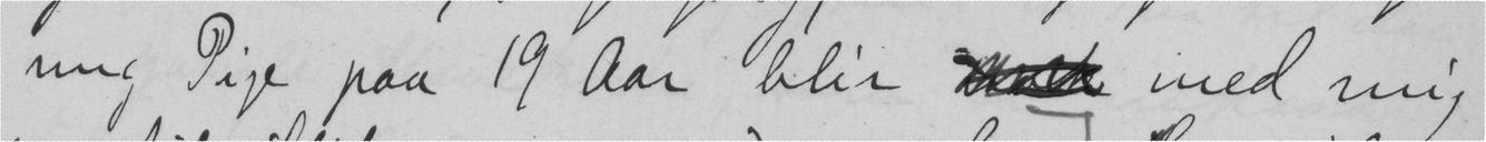ung Pige paa 19 Aar blir  med mig
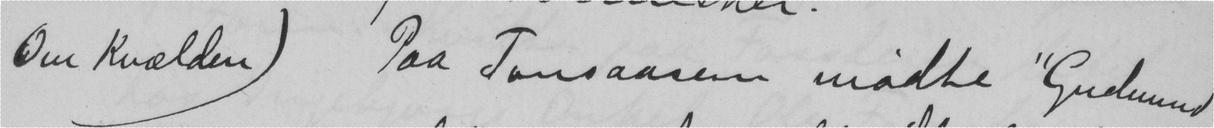Om Kvælden) Paa Tonsaasen mødte "Gudmund
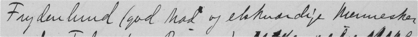Frydenlund (god Mad og elskværdige mennesker
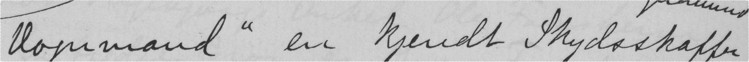Vognmand" en kjendt Skydsskaffer
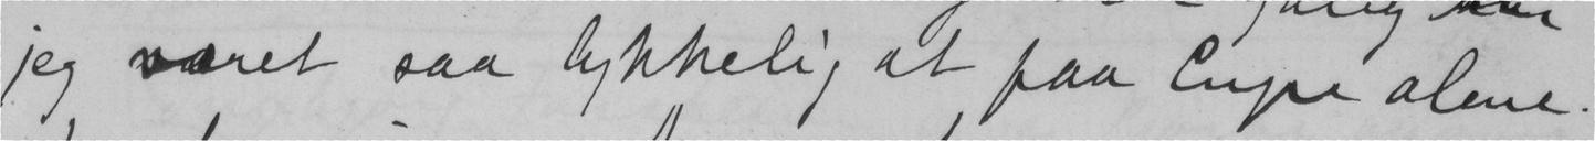jeg været saa lykkelig at faa Cupe alene.
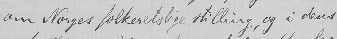om Norges folkeretslige stilling, og i dens
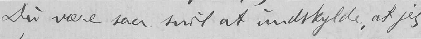Du være saa snil at undskylde, at jeg
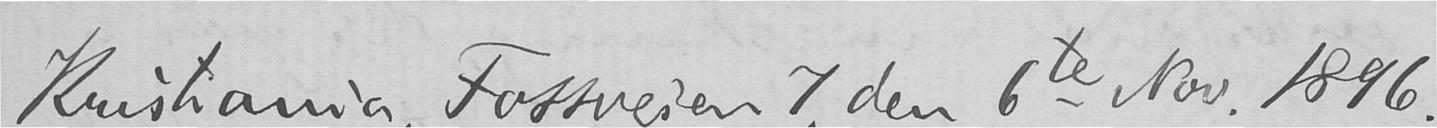Kristiania, Fossveien 7, den 6te Nov. 1896.
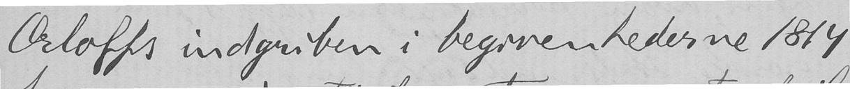Orloffs indgriben i begivenhederne 1814
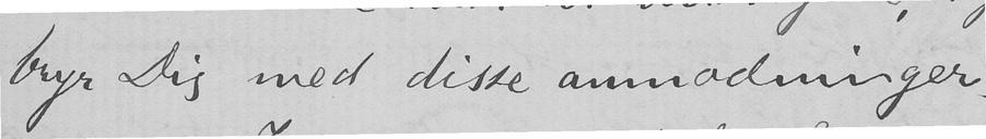bryr Dig med disse anmodninger.
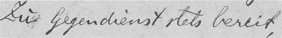Zu Gegendienst stets bereit,
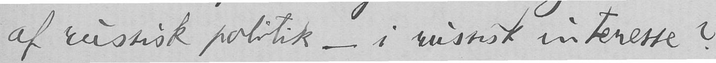af russisk politik - i russisk interesse?
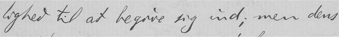lighed til at begive sig ind; men dens
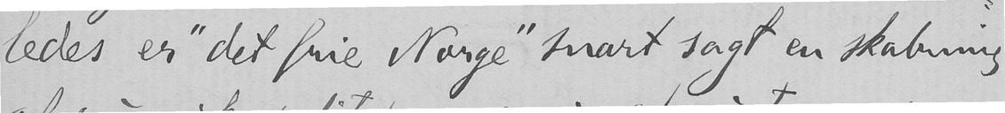ledes er "det frie Norge" snart sagt en skabning
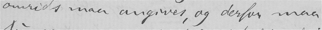omrids maa angives, og derfor maa
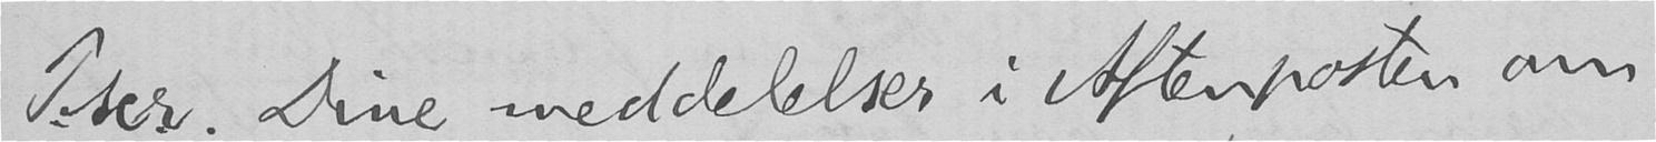P. scr. Dine meddelelser i Aftenposten om
